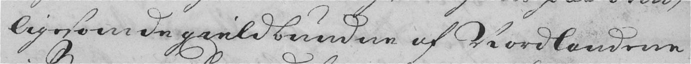ligesom de gieldbundne af Nordlandene
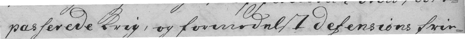passerede Krig, og formedelst defensions frie-
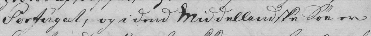Portugal, og i dend Middellandske Søe er
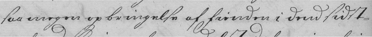saa megen opbringelse af Fienden i dend sidst-
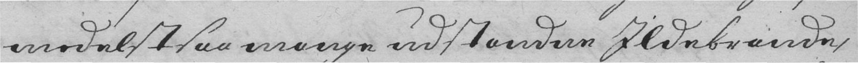medelst saa mange udstandne Ildebrande,
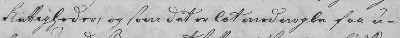Rettigheder, og som det er læt med nogle faa u-
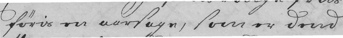føris en aarsage, som er dend
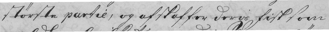største partie, og afskaffer deris Fisk som
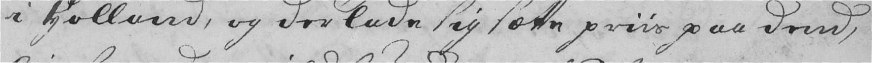i Holland, og der lade sig sætte priis paa dend,
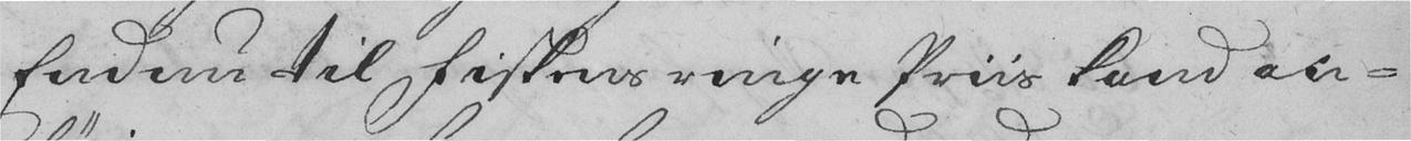Endnu til Fiskens ringe Priis kand an-
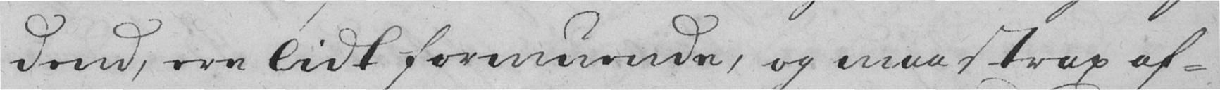dend, ere lidt formuende, og maa strax af-
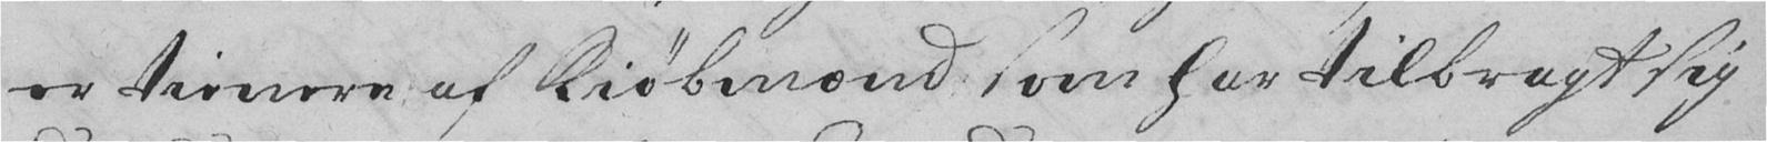er tienere af Kiøbmænd som har tilbragt sig
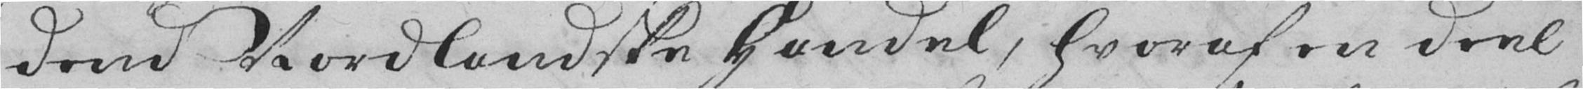dend Nordlandske Handel, hvoraf en deel
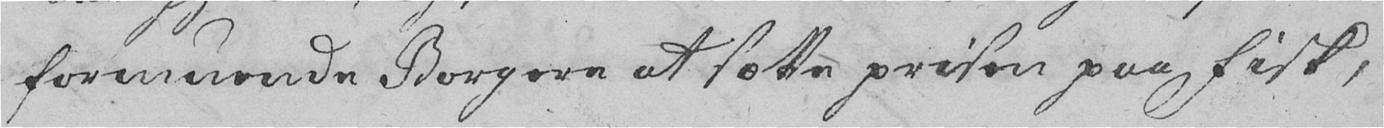formuende Borgere at sætte prisen paa Fisk,
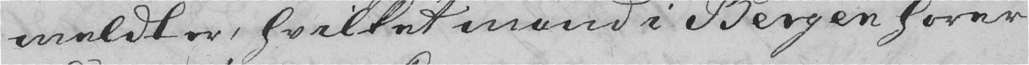meldt er, hvilket mand i Bergen hører
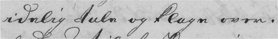idelig tale og klage over.
